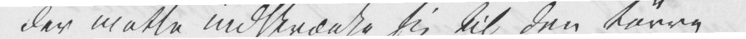der måtte indskrænke sig til den tørre
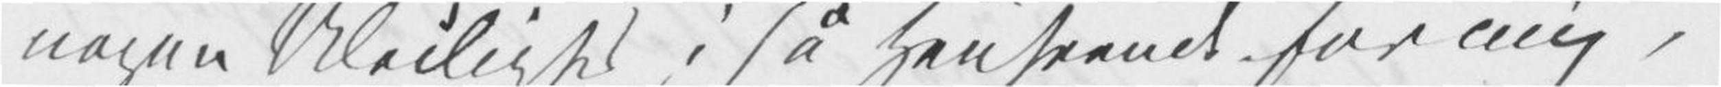nogen Uleilighed i så Henseende for mig,
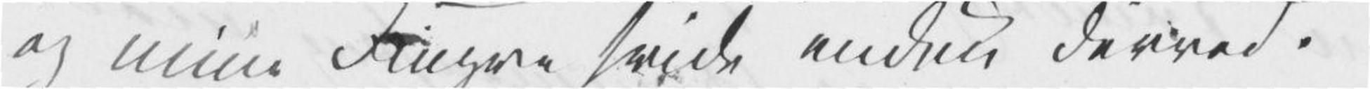og mine Fingre svide endnu derved.
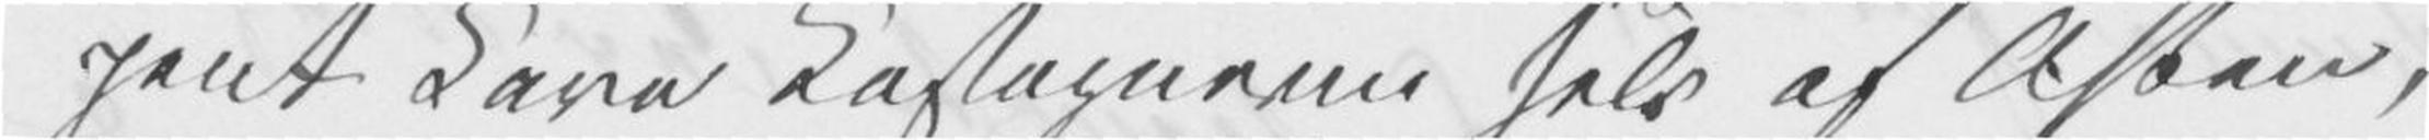pent kare Kastagnene selv af Asken,
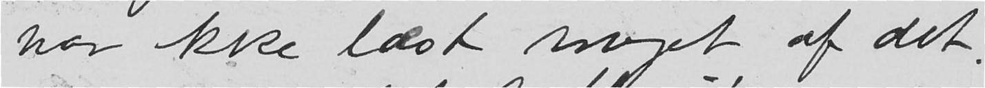har ikke læst noget af det.
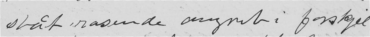ståt rasende angreb i forskjel
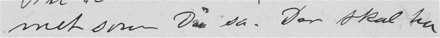met som Du sa. Der skal hu
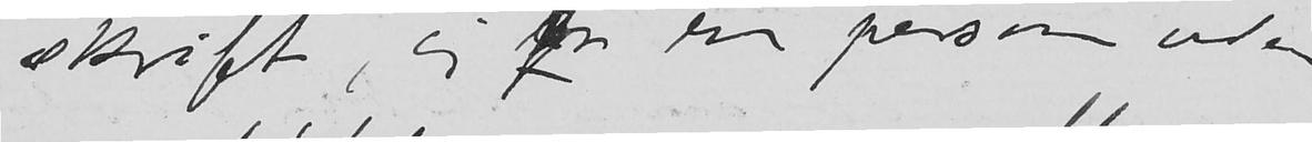skrift, og er en person uden
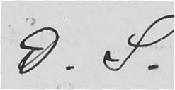D.S.
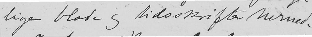lige blade og tidsskrifter hernede.
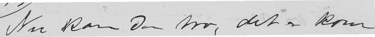Nu kan Du tro, det er kom-
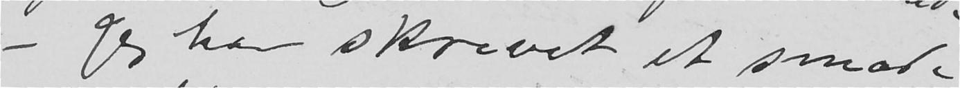- Jeg har skrevet et smæde
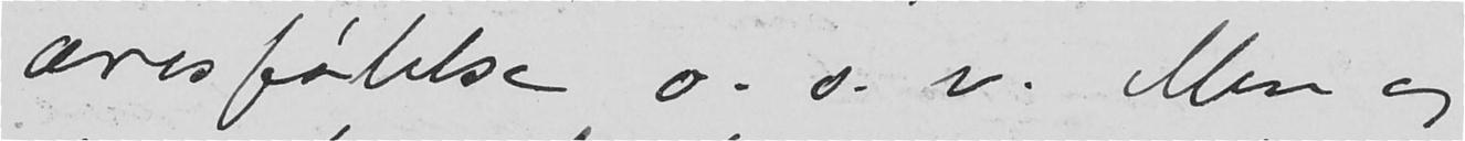æresfølelse o.s. v. Men jeg
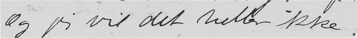Og jeg vil det heller ikke.
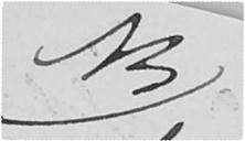NB)
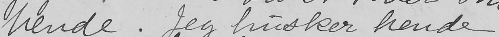hende. Jeg husker hende
